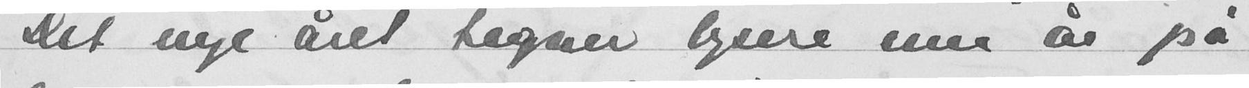Det nye året tegner lysere enn år på
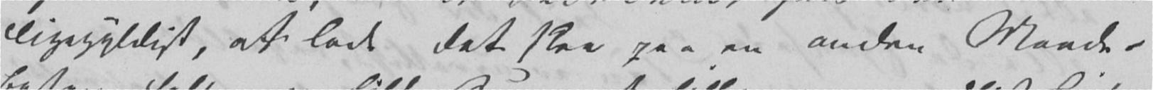ligegyldigt, at lade det skee paa en anden Maade –
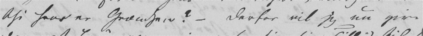thi hvor er Grændsen? – Derfor vil jeg nu give
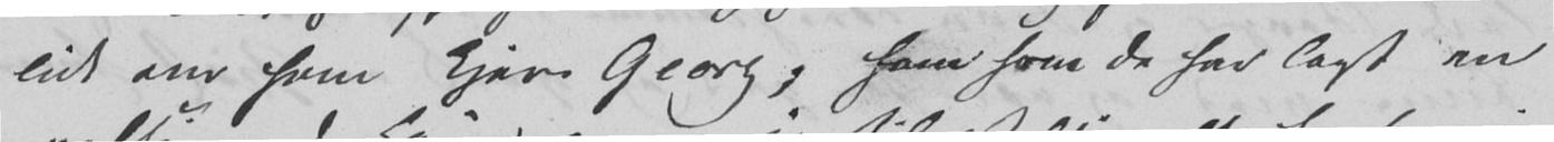lidt om ham kjere Georg; ham som De har lagt en
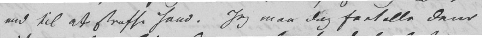end til at straffe ham. Jeg maa dog fortælle Dem
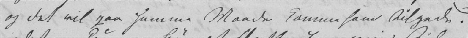og det vil paa samme Maade komme ham tilgode.
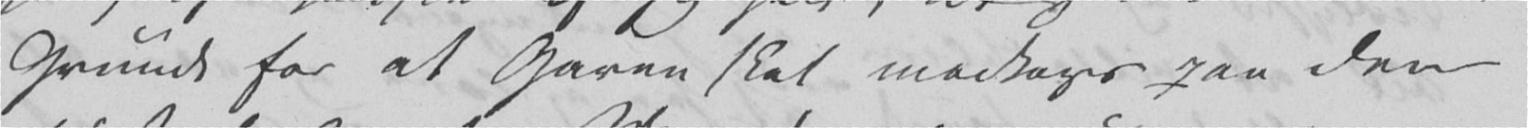Grunde for at Gaven skal modtages paa den
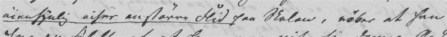øiensynlig viser en større Flid paa Skolen, røber at han
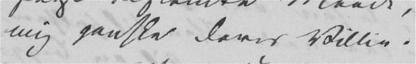mig ganske Deres Villie.
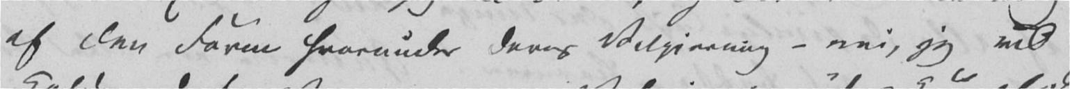af den Form hvorunder Deres Velgjerning – nei, jeg vil
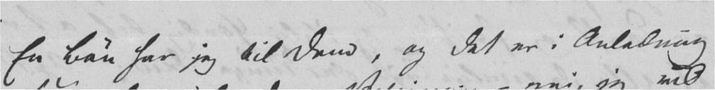En Bøn har jeg til Dem, og det er i Anledning
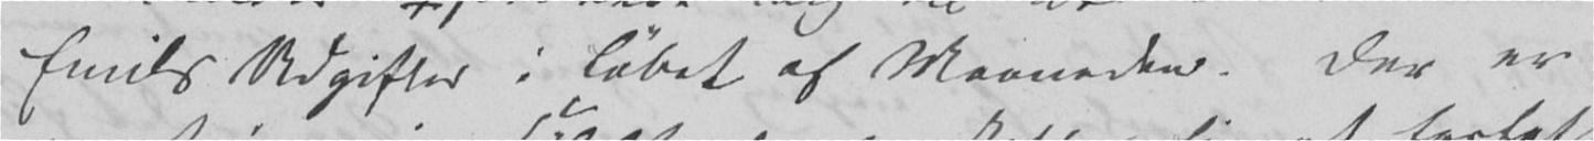Emils Udgifter i Løbet af Maaneden. Der er
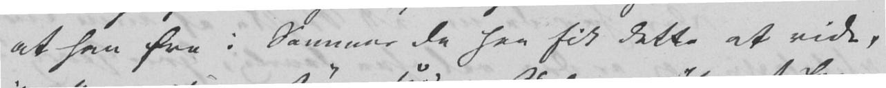at han fra i Sommer da han fik dette at vide,
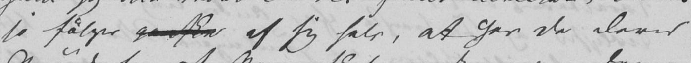jo følger ganske af sig selv, at har De Deres
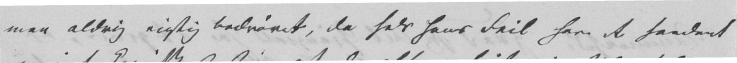men aldrig rigtig bedrøvet, da selv hans Feil have et saadant
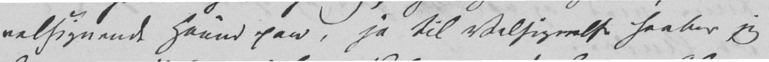velsignende Haand paa, ja til Velsignelse haaber jeg
